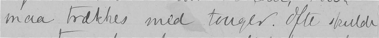maa trækkes med tauger. Ofte skulde
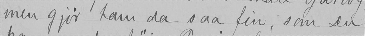men gjør ham da saa fin, som Du
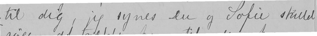til dig, jeg synes Du og Sofie skulde
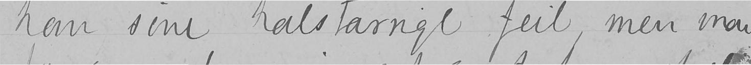han sine halstarrige feil, men man
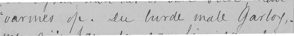varmes op. Du burde male Garborg, -
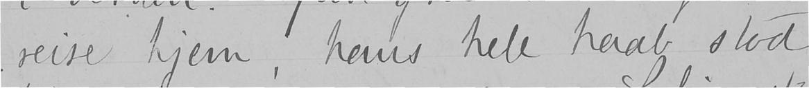reise hjem, hans hele haab stod
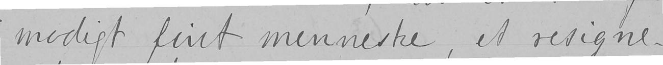modigt fint menneske, et resigne-
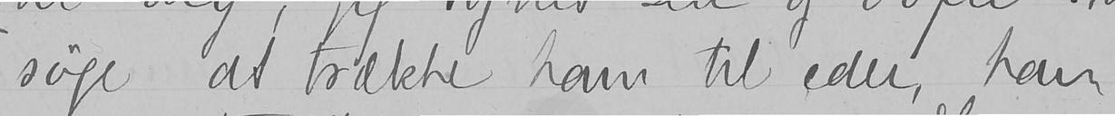søge at trække ham til eder, han
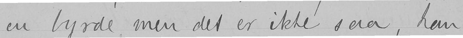en byrde, men det er ikke saa, han
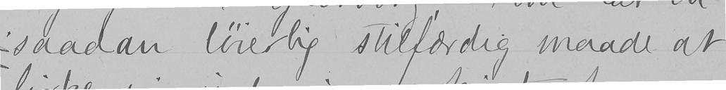saadan løierlig stilfærdig maade at
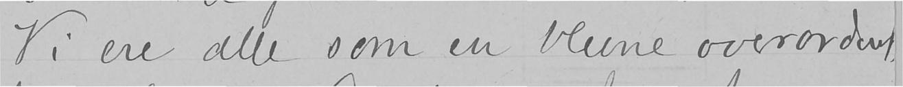Vi ere alle som en blevne overordent-
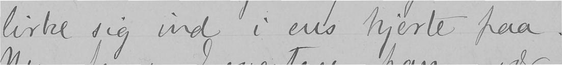lirke sig ind i ens hjerte paa.
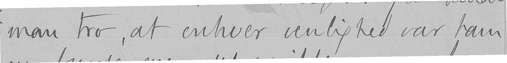man tro, at enhver venlighed var ham
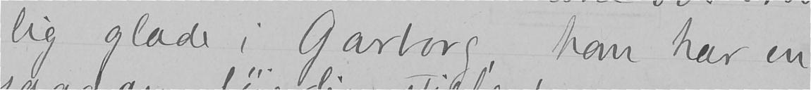lig glade i Garborg, han har en
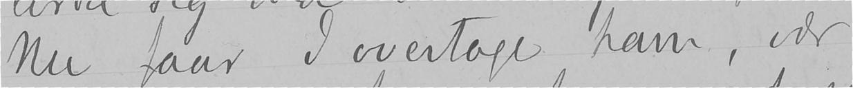Nu faar I overtage ham, vær
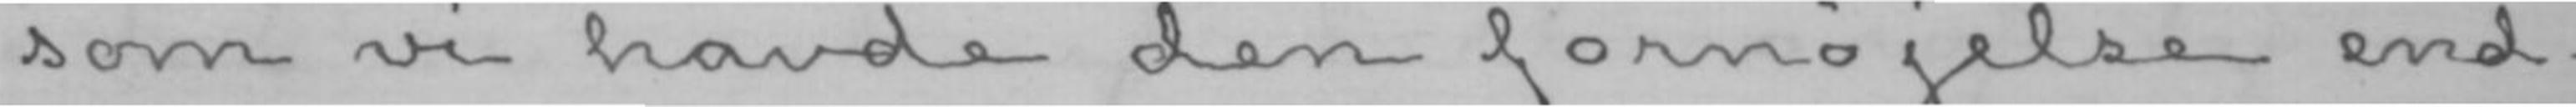som vi havde den fornøjelse end-
